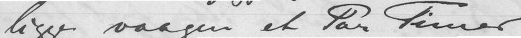ligge vaagen et Par Timer
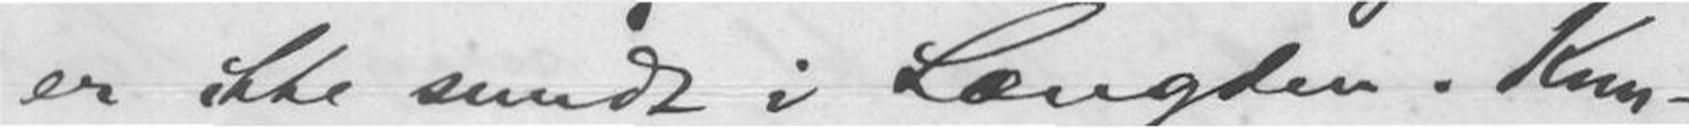er ikke sundt i Længden. Kun-
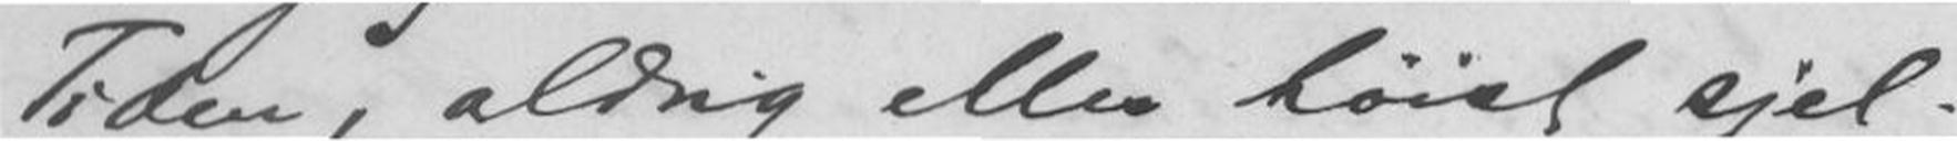Tiden, aldrig eller høist sjel-
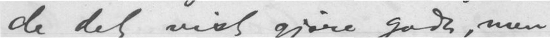de det vist gjøre godt, men
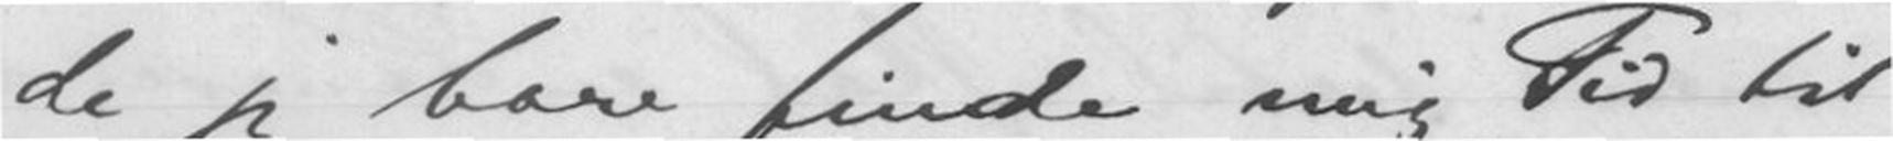de jeg bare finde mig Tid til
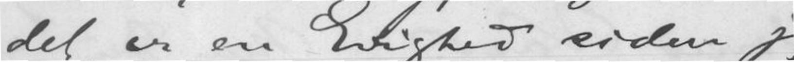det er en Evighed siden jeg
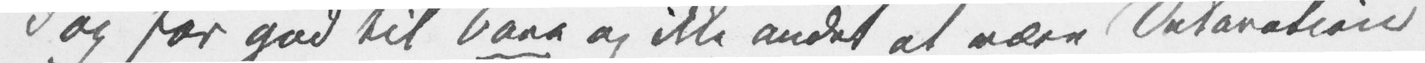dog for god til bare og ikke andet at være Dekoration
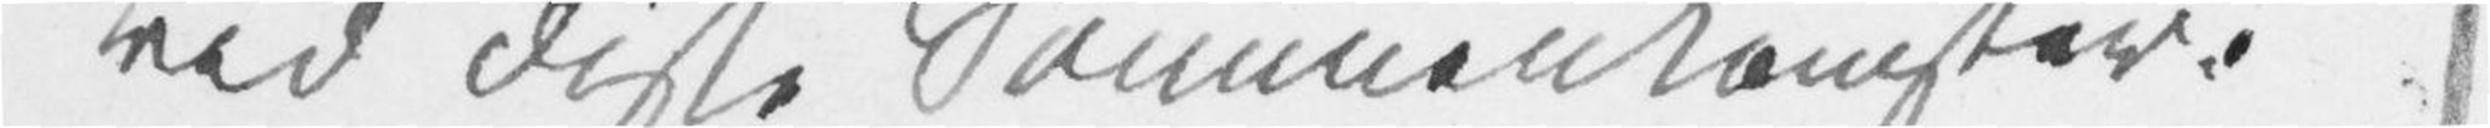ved disse Sammenkomster.
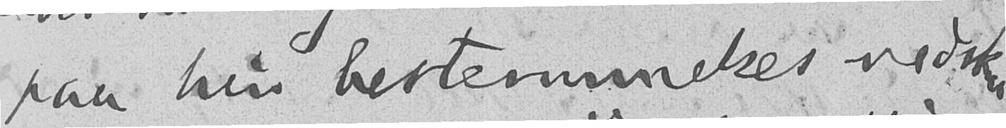paa hin bestemmelses nedskri-
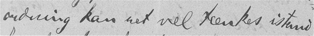ordning kan ret vel tænkes istand-
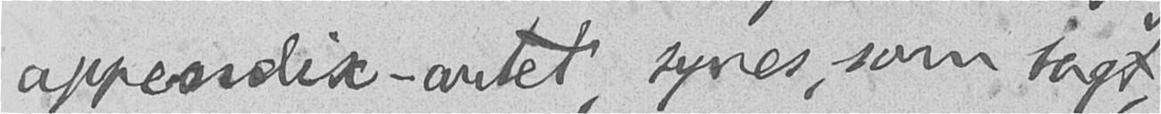appendix-artet, synes, som sagt,
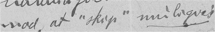mod, at "skip" muligvis
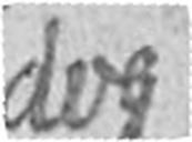dog
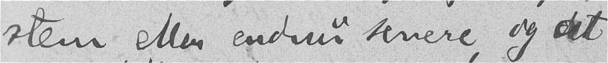stem eller endnu senere, og at
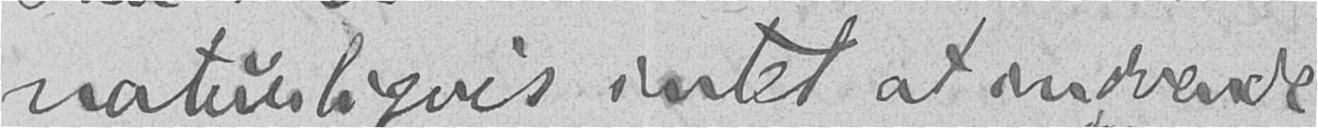naturligvis intet at indvende
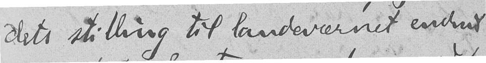dets stilling til landeværnet endnu
